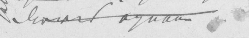derved opnaae
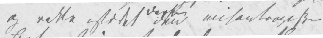og vakte istædet den misantropiske
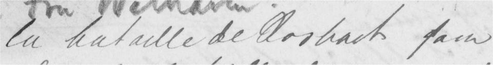La bataille de Rosbach som
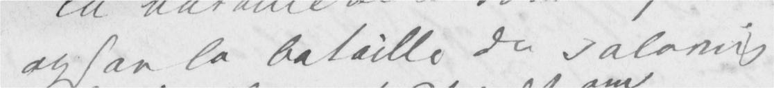ogsaa la bataille do Salamis
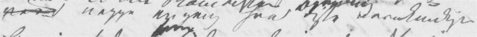veed neppe engang hvad disse navnkundige
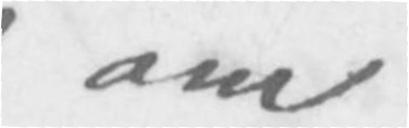om
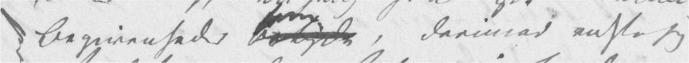Begivenheder betyde, derimod vidste jeg
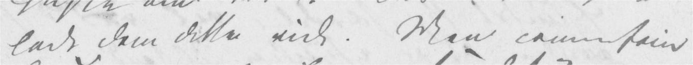lade Dem dette vide. Men <...>
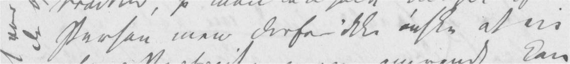en Person men derfor ikke ønske at eie
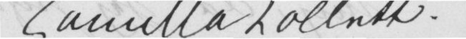Camilla Collett.
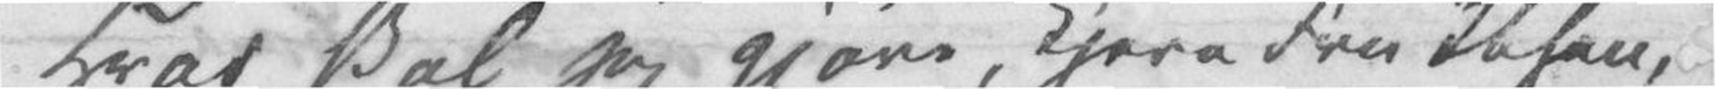Hvad skal jeg gjøre, kjere Fru Ibsen,
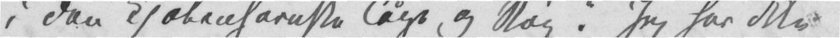i den kjøbenhavnske Tåge og Røg? Jeg har ikke
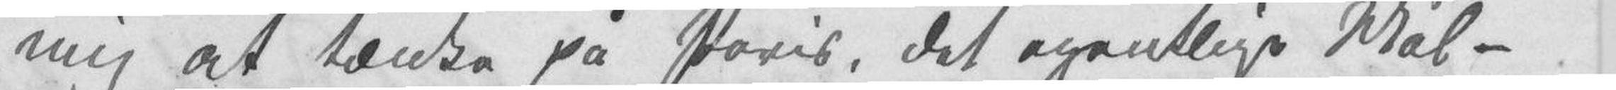mig at tænke på Paris, det egentlige Mål –
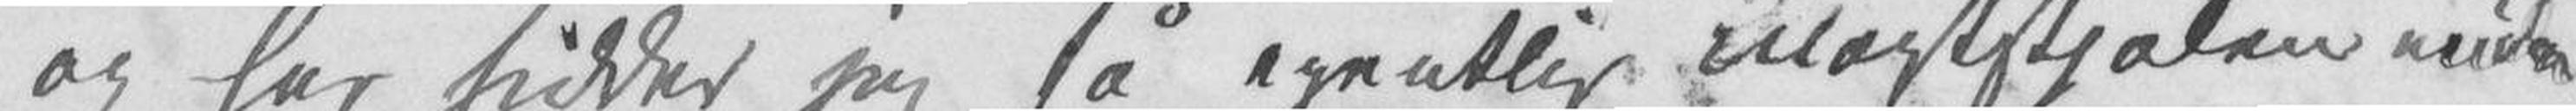og her sidder jeg så egentlig Magtstjålen endnu
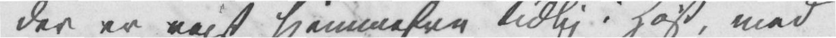der er reist hjemmefra tidlig i Høst, med
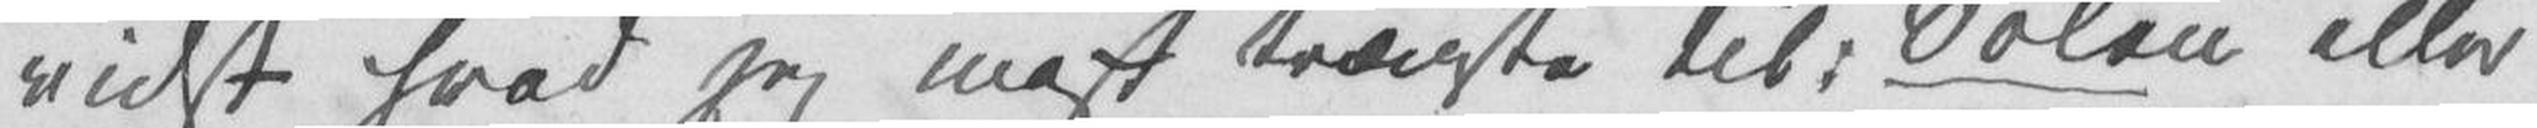vidst hvad jeg mest trængte til: Solen eller
